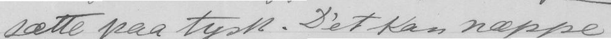sætte paa tysk. Det kan næppe
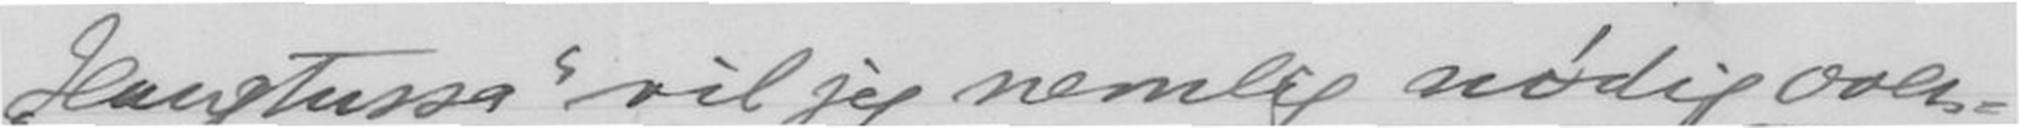"Haugtussa! vil jeg nemlig nødig over-
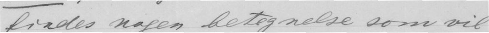findes nogen betegnelse som vil
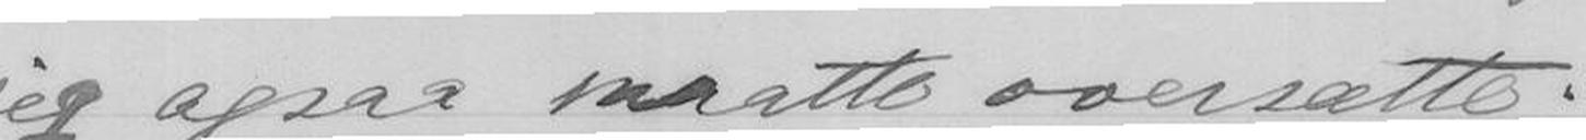jeg ogsaa maatte oversætte.
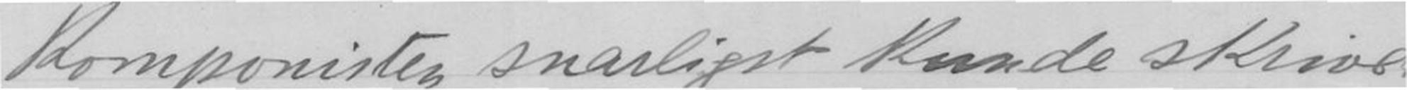Komponisten snarligt kunde skrive mig
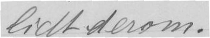lidt derom.
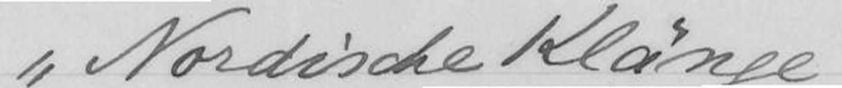"Nordiske Klänge"
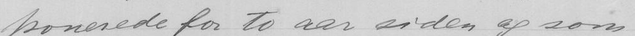ponerede for to aar siden og som
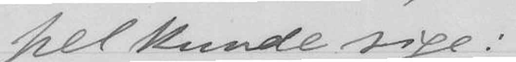pel kunde sige:
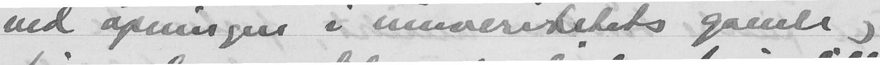ved åpningen i muaeriketets gamle og
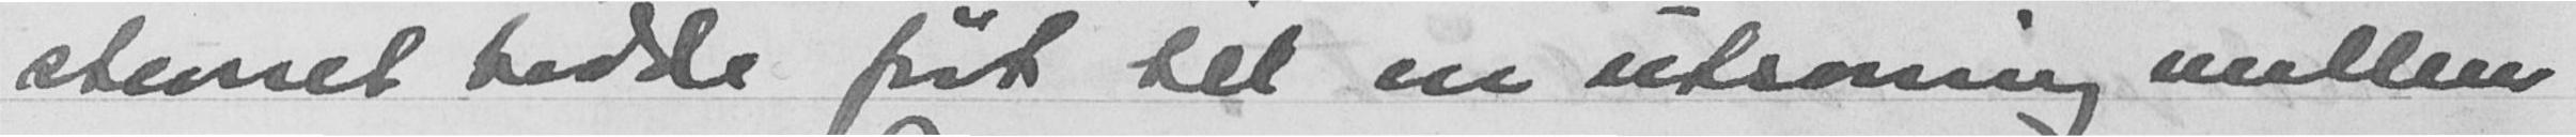stresset hadde ført til en utsoning mellom
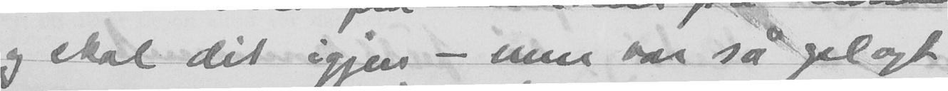og skal dit igjen - men var så oplagt og
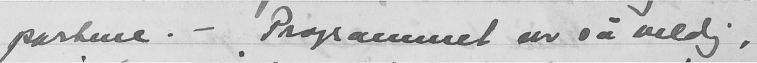partene. - Programmet var så veldig,
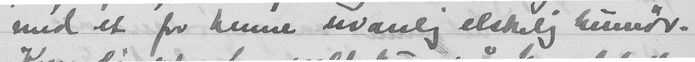med et for henne uvanlig elskelig humør.
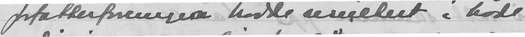fortatterforeningene hadde resultert i både
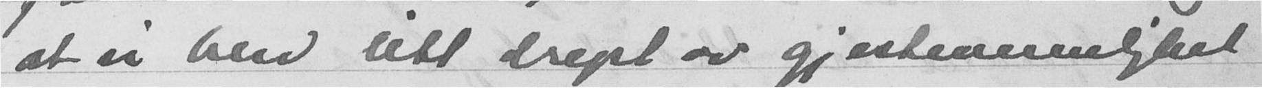at vi blir litt drept av gjestevennlighet
